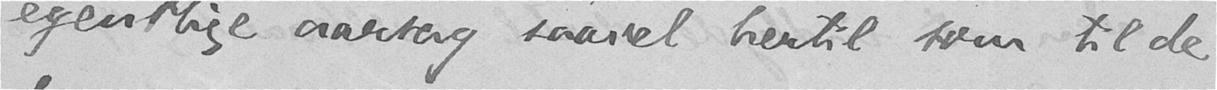egentlige aarsag saavel hertil som til de
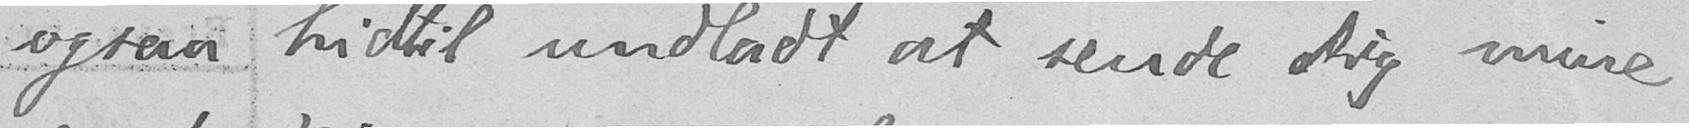ogsaa hidtil undladt at sende Dig mine
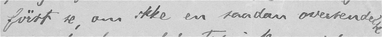først se, om ikke en saadan oversendelse
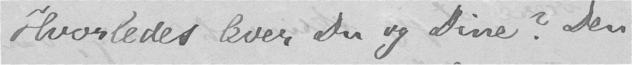Hvorledes lever Du og Dine? Den
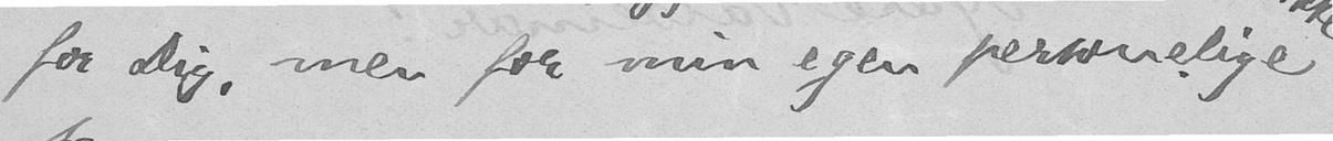for Dig, men for min egen personelige
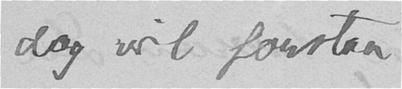dog vil forstaa.
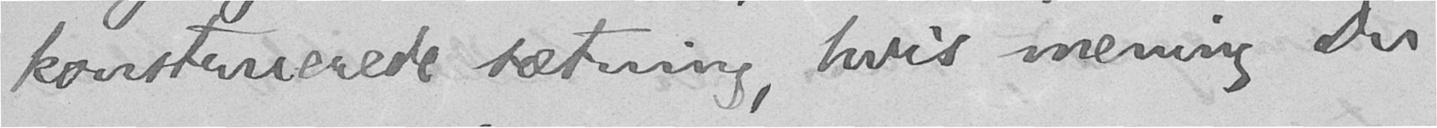konstruerede sætning, hvis mening Du
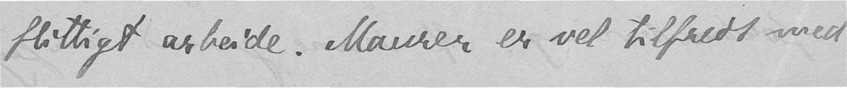flittigt arbeide. Maurer er vel tilfreds med
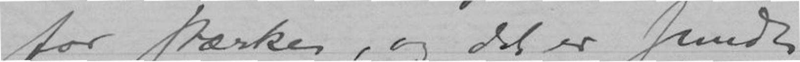for stærke, og det er sundt
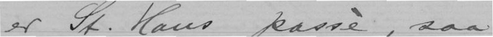er St. Hans passè, saa
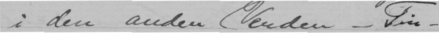i den anden Verden - Tin-
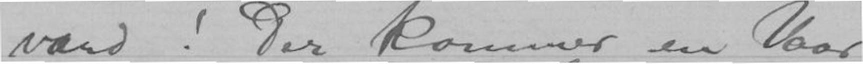vard! Der kommer en Vaar
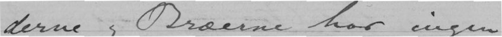derne og Bræerne har ingen
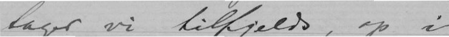tager vi tilfjelds, op i
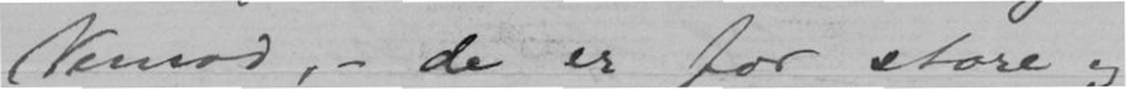Vemod, - de er for store og
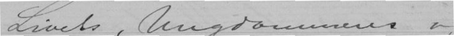Livets, Ungdommens og
